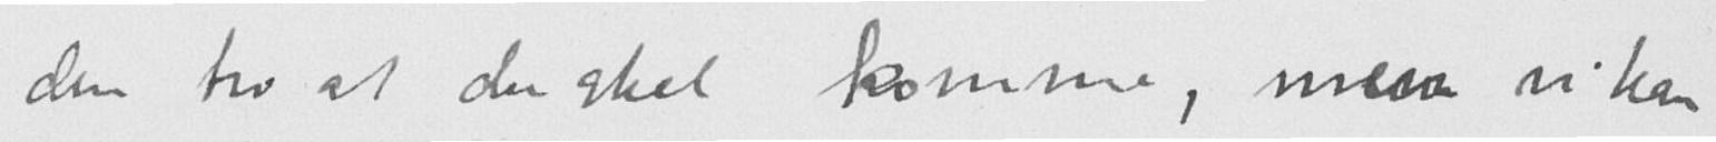den tro at du skal komme, men vi kan
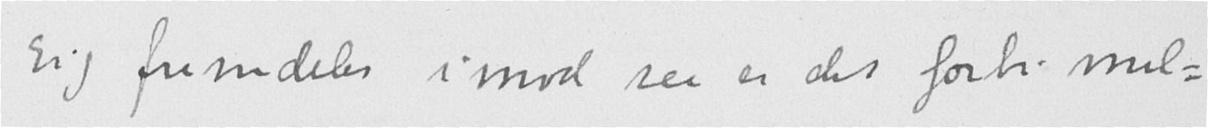sig fremdeles i mod saa er det forbi mel-
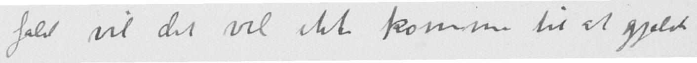fald vil det vel ikke komme til at gjælde
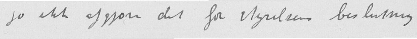jo ikke afgjøre det før styrelsens beslutning
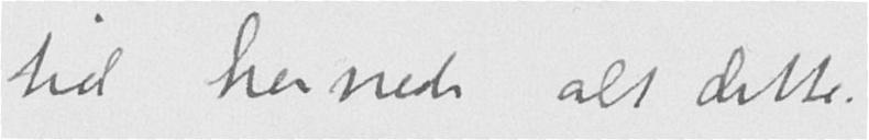tid hernede alt dette.
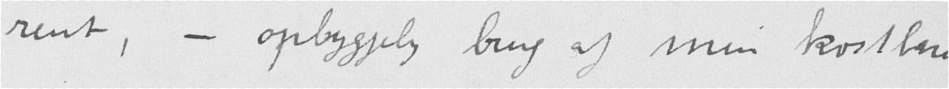rent, - opbyggelig brug af min kostbare
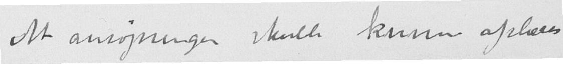At ansøgningen skulde kunne afslaaes
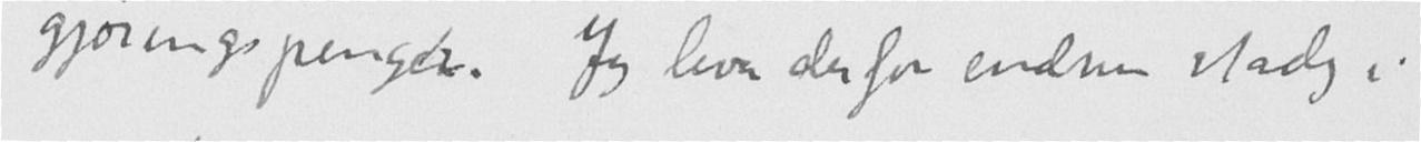gjøringspenger. Jeg lever derfor endnu stadig i
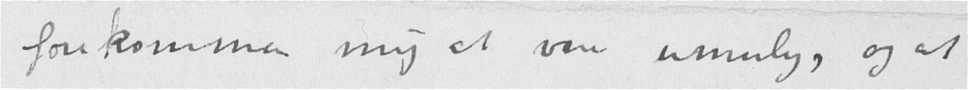forekommer mig at være umulig, og at
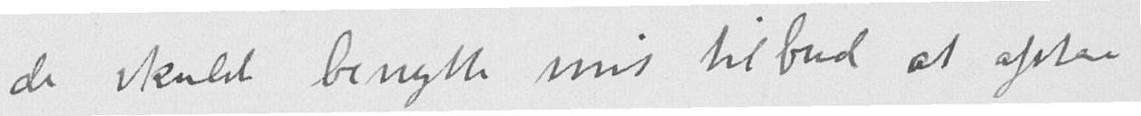de skulde benytte mit tilbud at afstaa
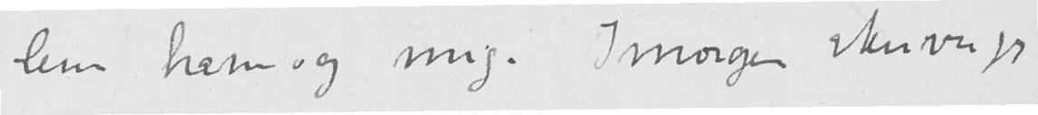lem ham og mig. I morgen skriver jeg
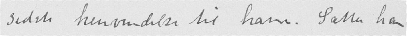sidste henvendelse til ham. Sætter han
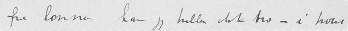fra lønnen kan jeg heller ikke tro - i hvert
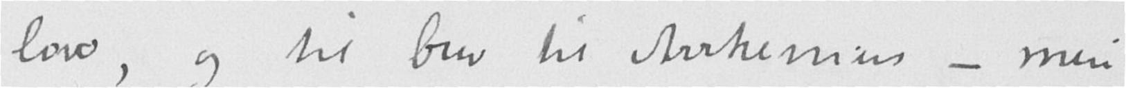low, og til brev til Arrhenius - min
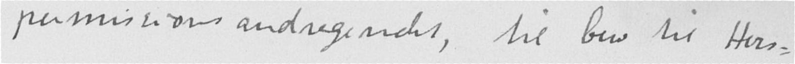permissionsandragendet, til brev til Hers-
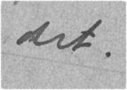det.
